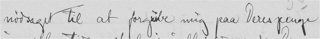nødsaget til at forgribe mig paa Deres penge
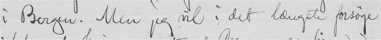i Bergen. Men jeg vil i det længste forsøge
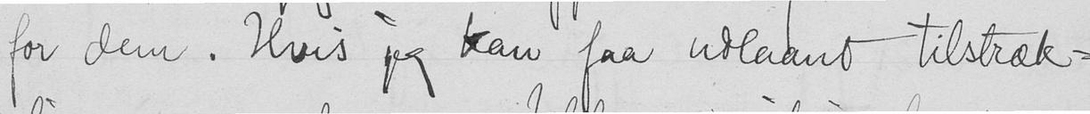for dem. Hvis jeg kan faa udlaant tilstræk-
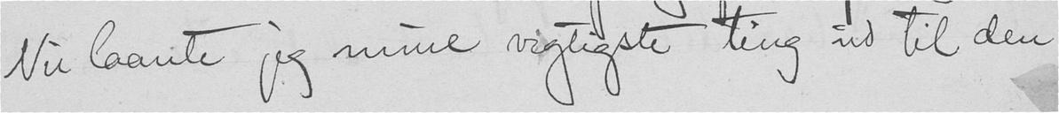Nu laante jeg mine vigtigste ting ud til den
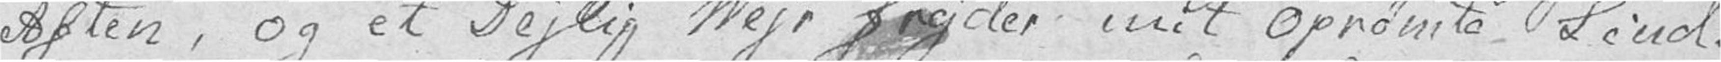Aften, og et Dejlig Vejr fryder mit Oprømte Sind.
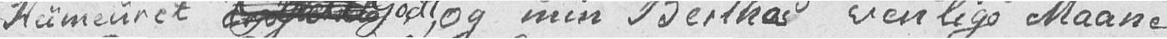Humeuret  Godt, og min Berthas venlige Maane
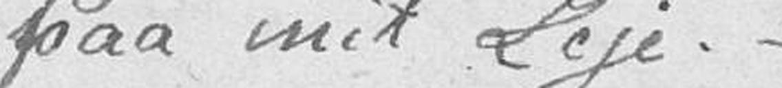paa mit Leje. –
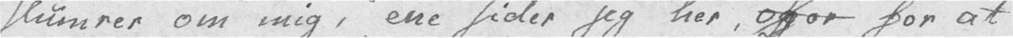slumrer om mig, ene sider jeg her, ofor for at
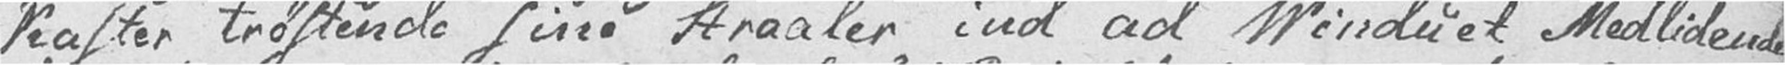kaster trøstende sine Straaler ind ad Vinduet Medlidende
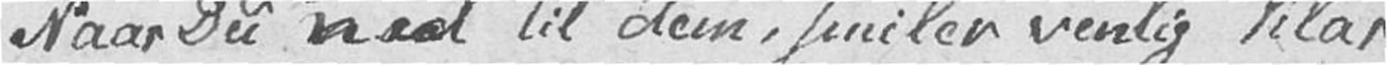Naar Du ned til dem, smiler venlig klar
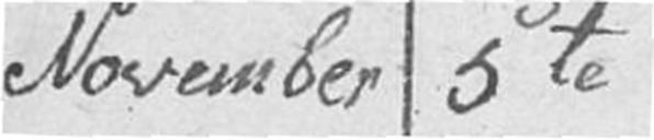November 5te
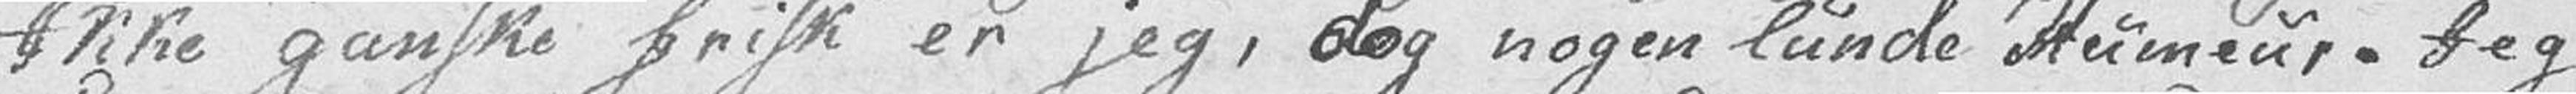Ikke ganske frisk er jeg, dog nogen lunde Humeur. Jeg
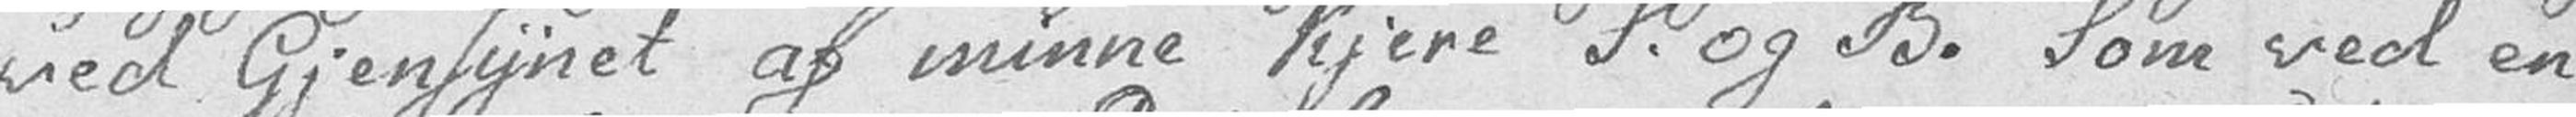ved Gjensynet af minne kjere S. og B. Som ved en
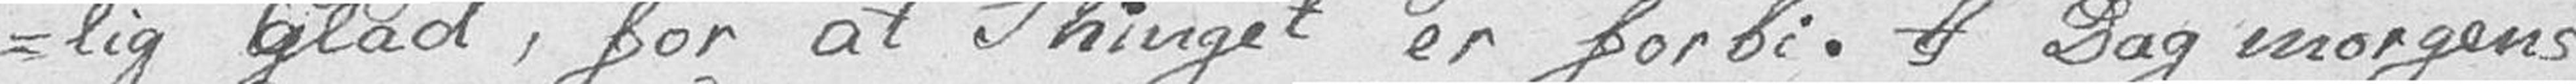-lig Glad, for at Thinget er forbi. I Dag morgens
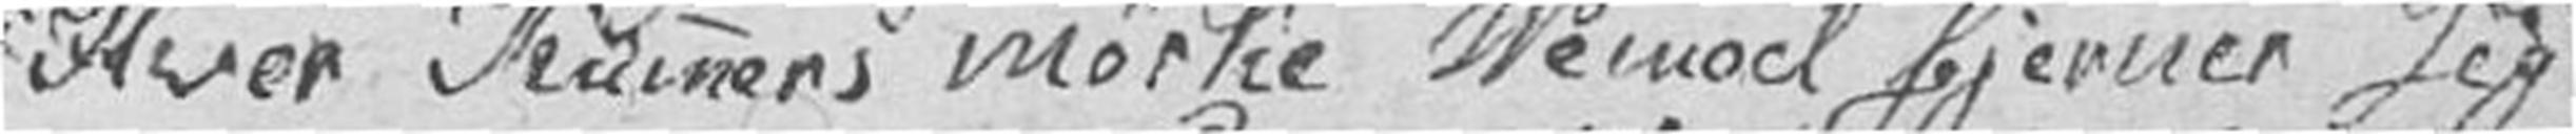Hvor Kummers mørke Vemod fjerner sig
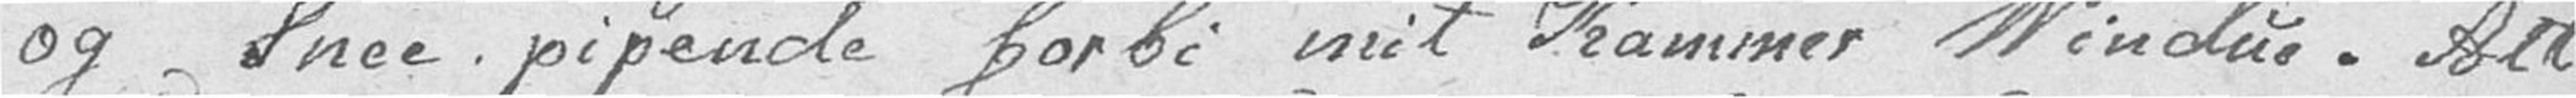og Snee pipende forbi mit Kammer Vindue. Alt
